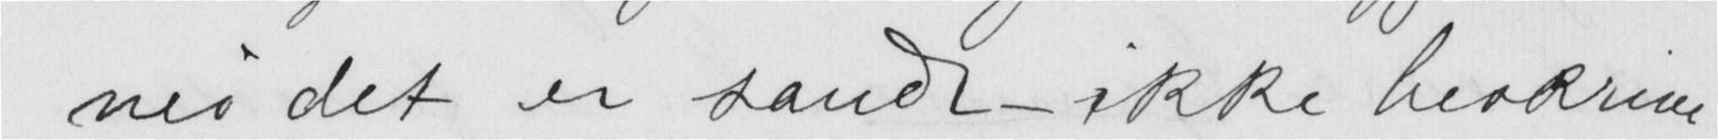nei det er sandt- ikke herkreve
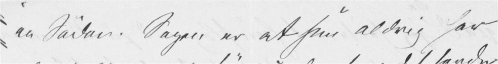en Sådan. Sagen er at hun aldrig har
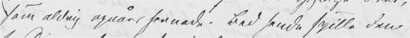som aldrig opnåes hernede. Bed hende spille den
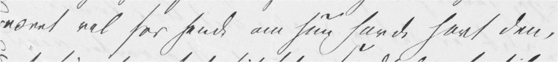været vel for hende om hun havde havt den,
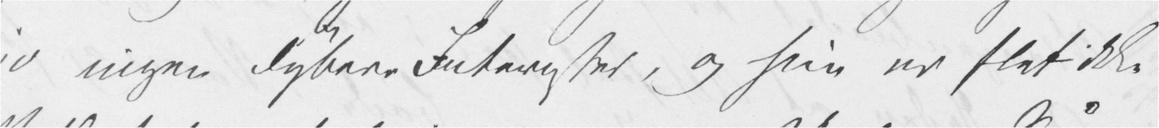jo ingen dybere Interesser, og hun er slet ikke
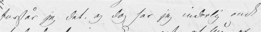forstår jeg det. og dog har jeg inderlig ondt
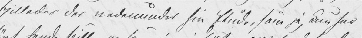spilledes der nedenunder hin Etude, som jeg kun har
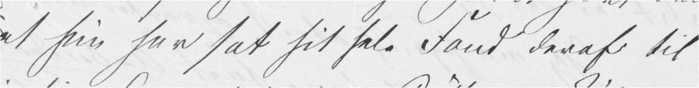at hun har sat sit hele Fond deraf til
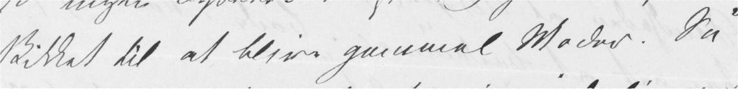skikket til at blive gammel Moder. Så
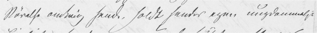Rørelse omkring hende, holdt hendes egen ungdommelige
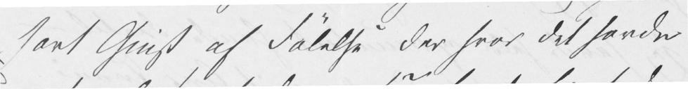havt Gnist af Følelse der hvor det havde
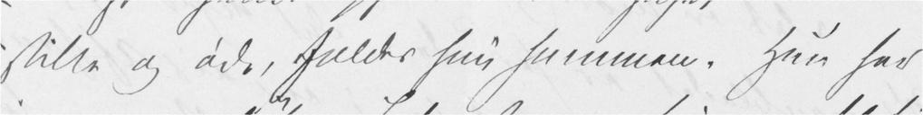stille og øde, falder hun sammen. Hun har
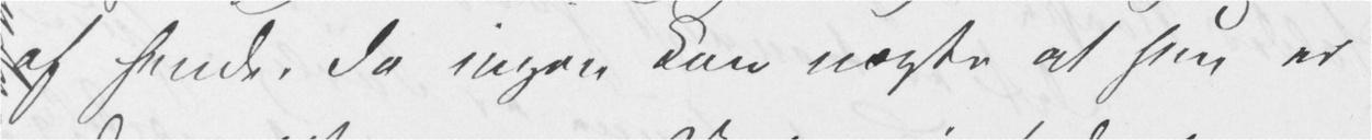af hende, da ingen kan nægte at hun er
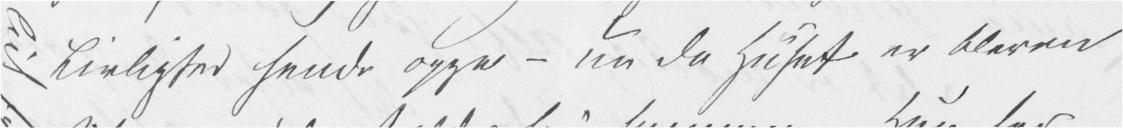Livlighed hende oppe – nu da Huset er bleven
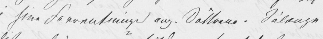i sine Forretninger ang. Døttrene. Sålænge
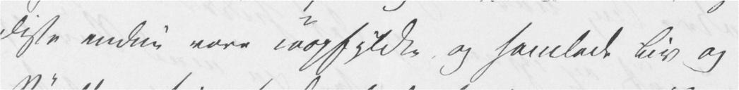disse endnu vare uopfyldte og samlede Liv og
